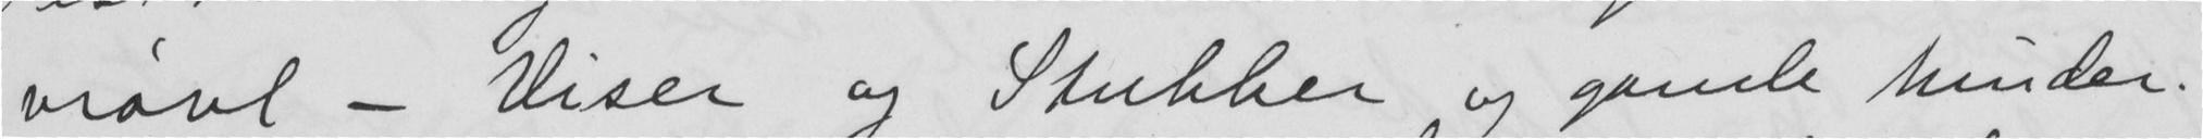vrøvl - Viser og Stubber og gamle Minder.
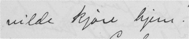vilde kjøre hjem.
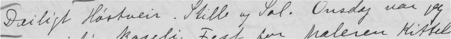Deiligt Høstveir. Stille og Sol. Onsdag var jeg
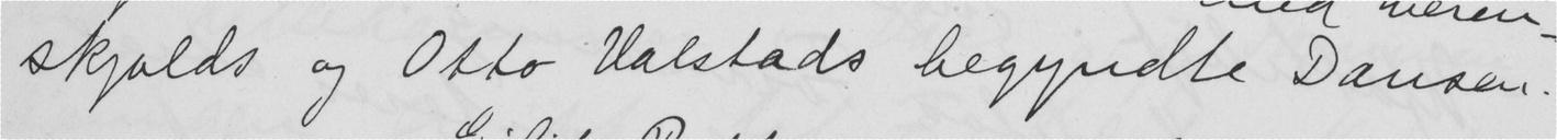skjolds og Otto Valstads begyndte Dansen.
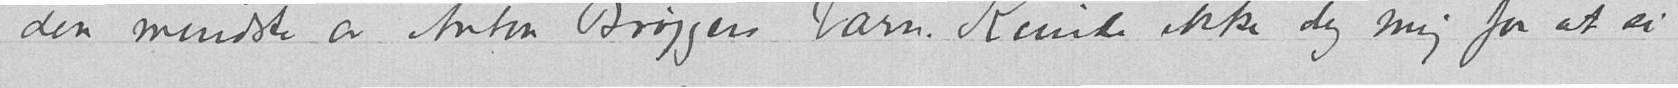den mindste av Anton Brøggers barn. Kunde ikke dy mig for at si
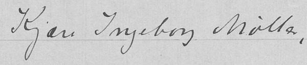Kjære Ingeborg Møller,
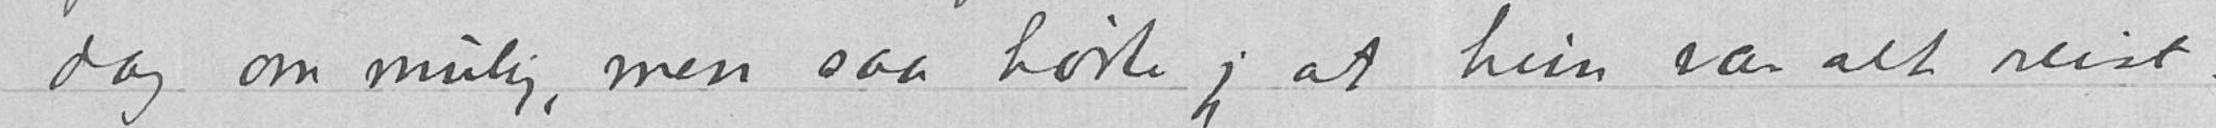dag om mulig, men saa hørte jeg at hun var alt reist.
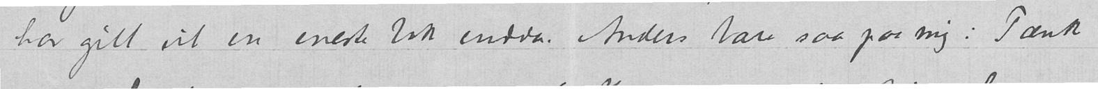har gitt ut en eneste bok endda. Anders bare saa paa mig: Tænk
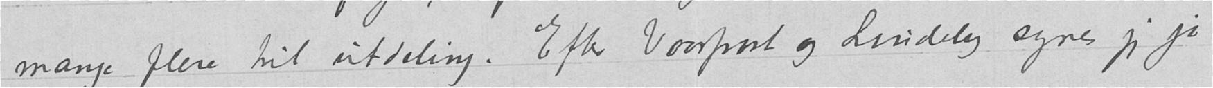mange flere til utdeling. Efter Vaarfrost og Lindeby synes jeg jo
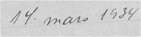14. mars 1934
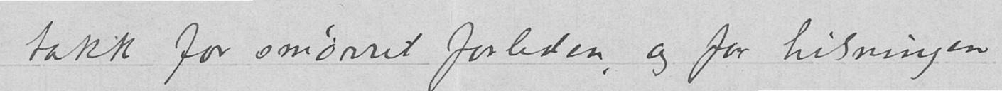takk for smørret forleden, og for hilsningen
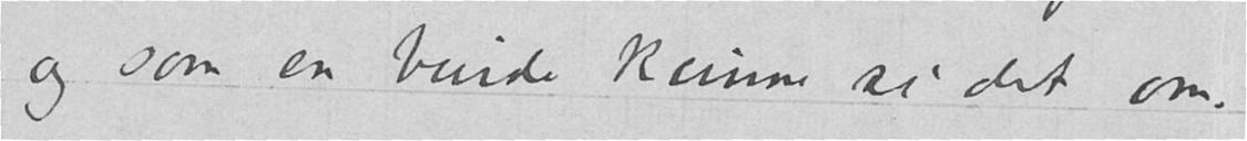og som en burde kunne si det om.
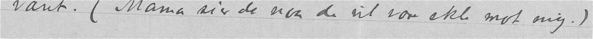været. (Mama sier de naar de vil være ækle mot mig.)
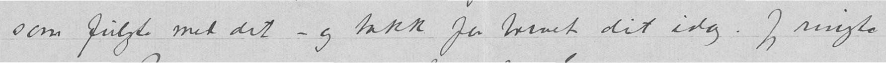som fulgte med det – og takk for brevet dit idag. Jeg ringte
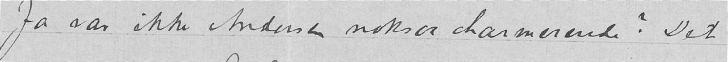Ja var ikke Andersen noksaa charmerende? Det
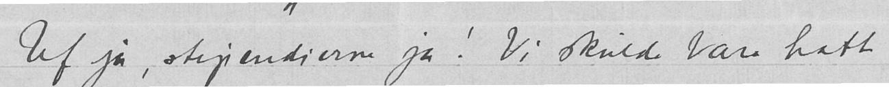Uf ja, stipendierne ja! Vi skulde bare hatt
